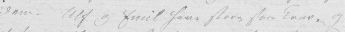dem. Alf og Emil have store, store Krav, og
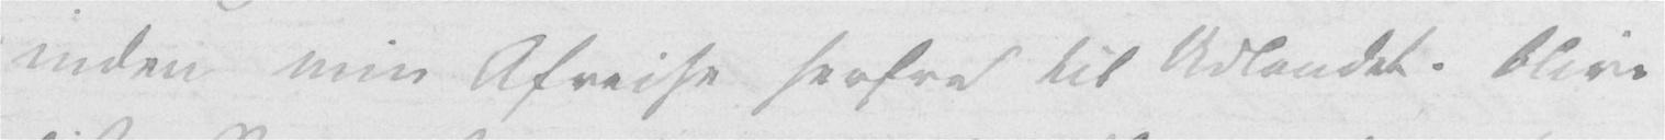inden min Afreise herfra til Udlandet. Blive
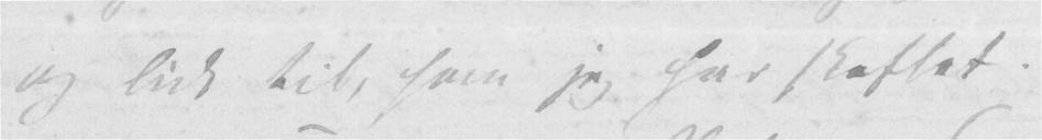og lidt til, som jeg har skaffet.
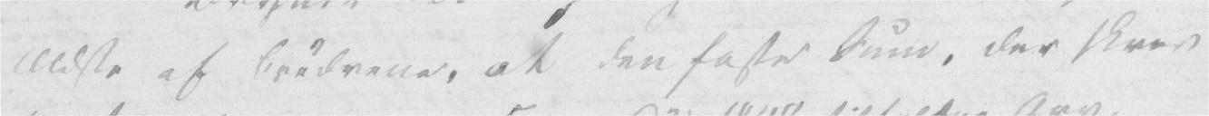Ældste af Brødrene, at den faste Sum, der skrev
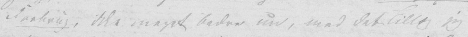Forbrug, ikke meget bedre nu, med det Tillæg jeg
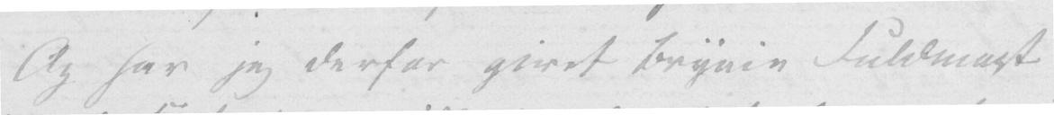Og har jeg derfor givet Brynie Fuldmagt
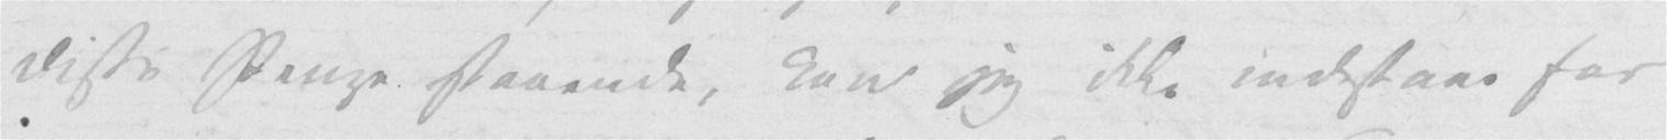disse Penge staaende, kan jeg ikke indestaae for
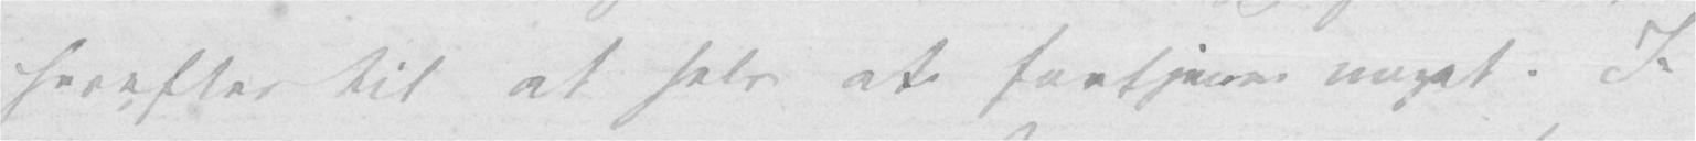herefter til at selv at fortjene noget. I
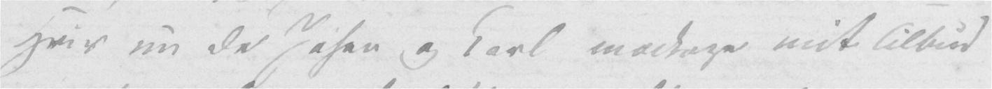Hvis nu De Johan og Carl modtage mit Tilbud
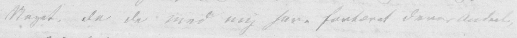Noget, da de med mig have fortæret deres Andeel,
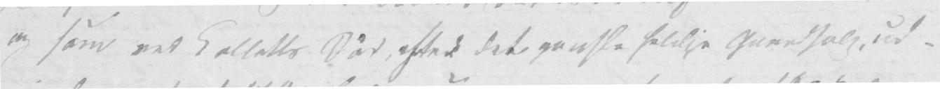og som ved Colletts Død, efter det ganske heldige Gaardsalg,
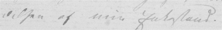af min Enkestand.
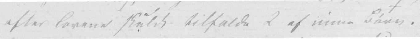efter Lovene skulde tilfalde 2 af mine Børn.
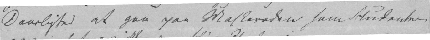Daarlighed at gaa paa Maskeraden som Studenterne
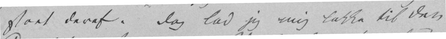stort deraf. Dog lod jeg mig lokke til den
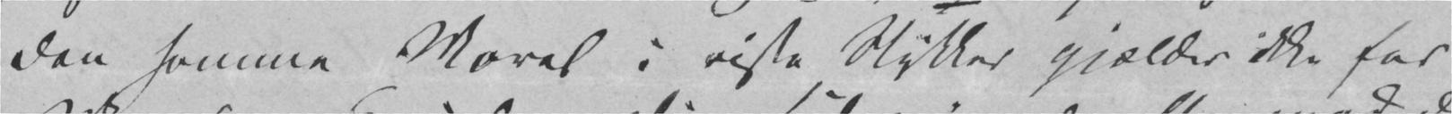den samme Moral i visse Stykker gjælder ikke for
